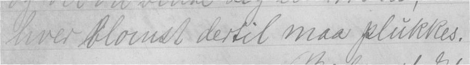hver blomst dertil maa plukkes."
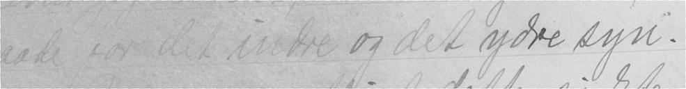baade for det indre og det ydre syn.
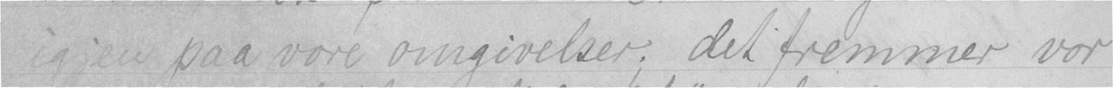igjen paa vore omgivelser; det fremmer vor
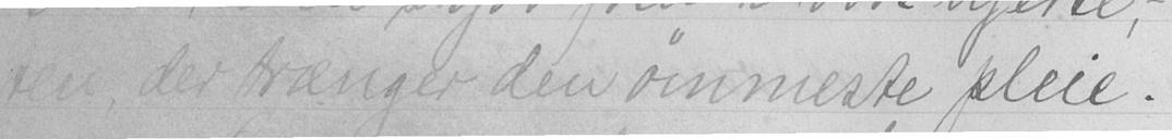ten, der trænger den ømmeste pleie.
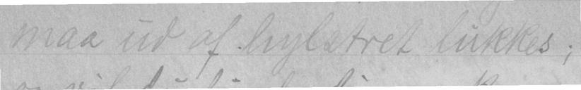maa ud af hylstret lukkes;
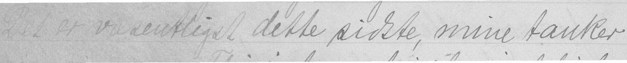Det er vesentligst dette sidste, mine tanker
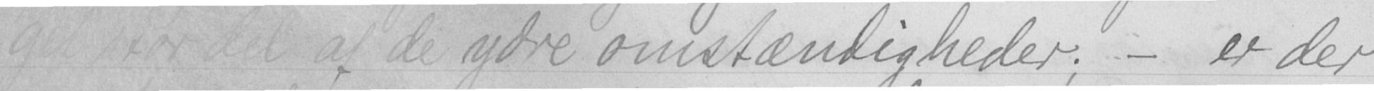gen stor del af de ydre omstændigheder; - er der
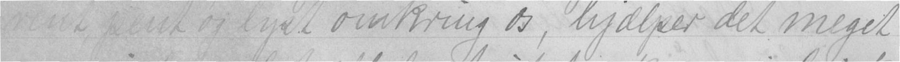rent, pent og lyst omkring os, hjælper det meget
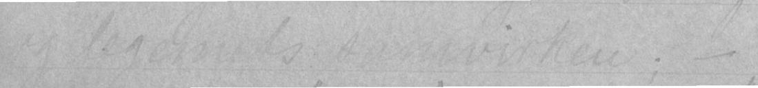og legemets samvirken; -
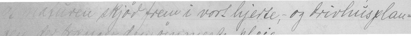i naturen skjød frem i vort hjerte, - og drivhusplan-
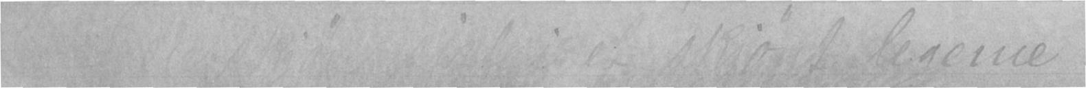skjønt legeme!
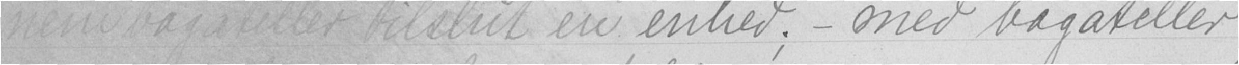nem bagateller tilslut en enhed; - med "bagateller"
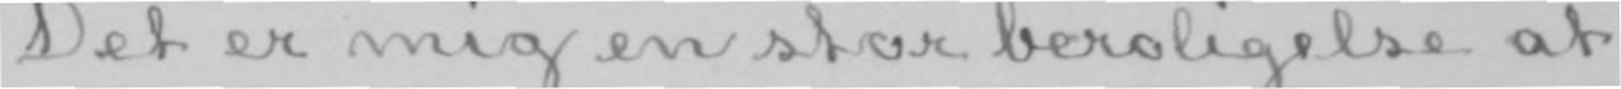Det er mig en stor beroligelse at
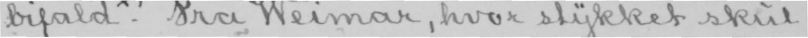bifald. Fra Weimar, hvor stykket skul-
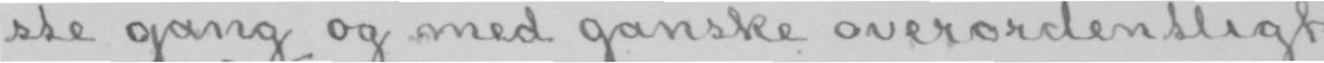ste gang og med ganske overordentligt
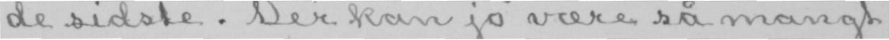de sidste. Der kan jo være så mangt
